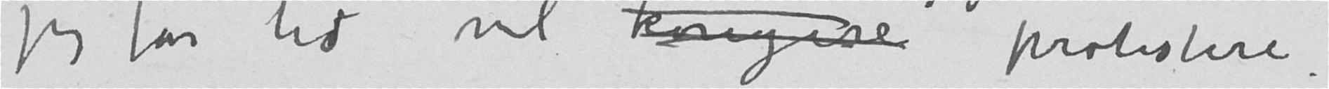jeg får tid vil korigere protestere.
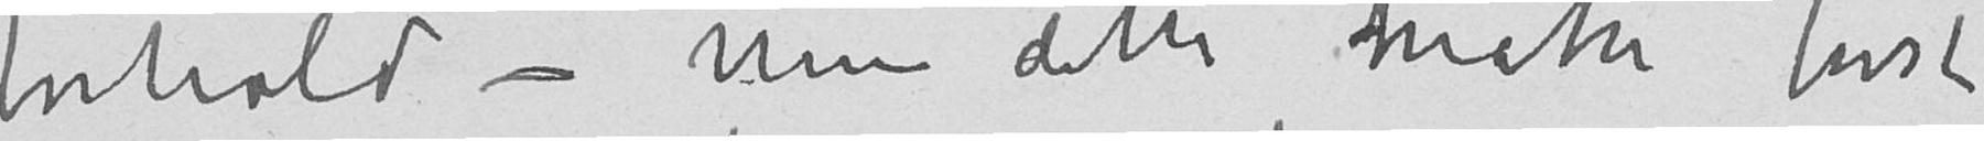forhold – Men dette måtte først
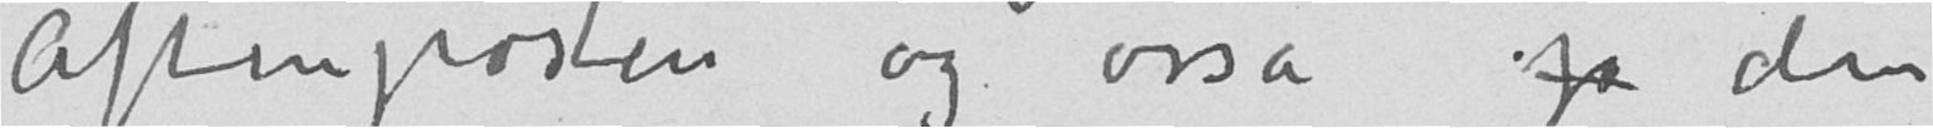Aftenposten og ossåden
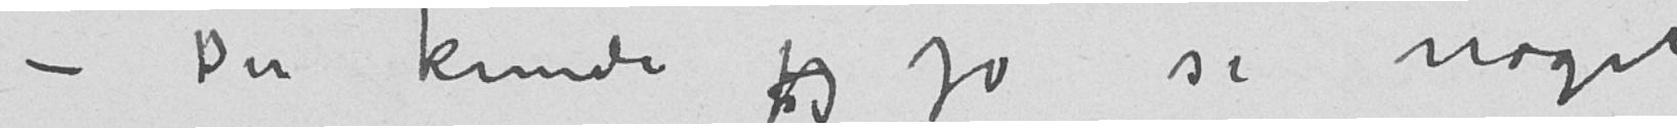– Du kunde jeg jo si noget
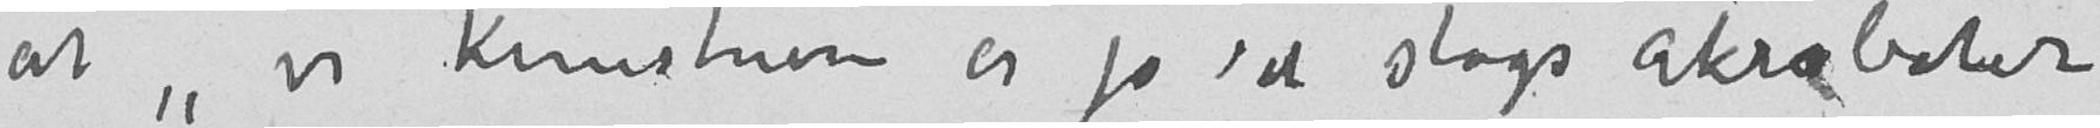at «vi Kunstnere er jo en slags akrobater
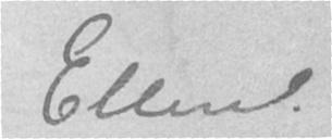Ellen.
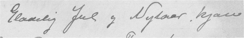Gledelig Jul og Nytaar. kjære
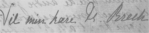Til min kære hr Broch
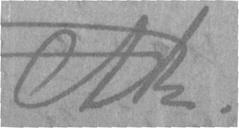A
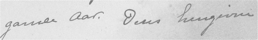gamle aar. Deres hengivne
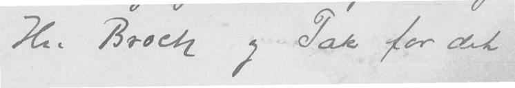Hr. Broch og Tak for det
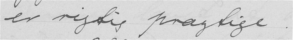er rigtig prægtige.
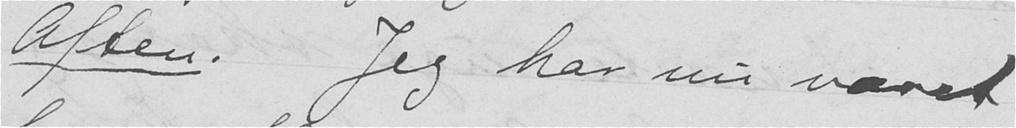Aften. Jeg har nu været
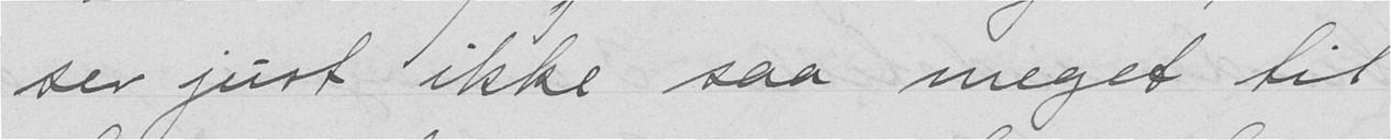ser just ikke saa meget til
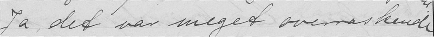Ja, det var meget overraskende
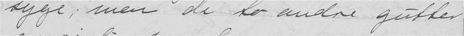syge; men de to andre gutter
